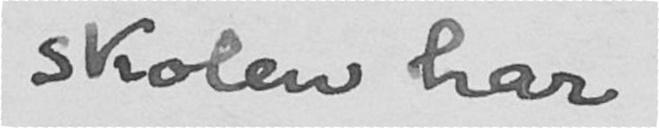skolen har
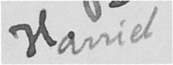Harriet
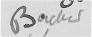Backer
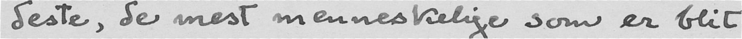deste, de mest menneskelige som er blit
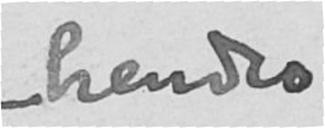hendes
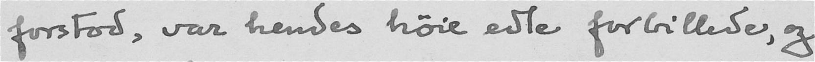forstod, var hendes høie edle forbillede, og
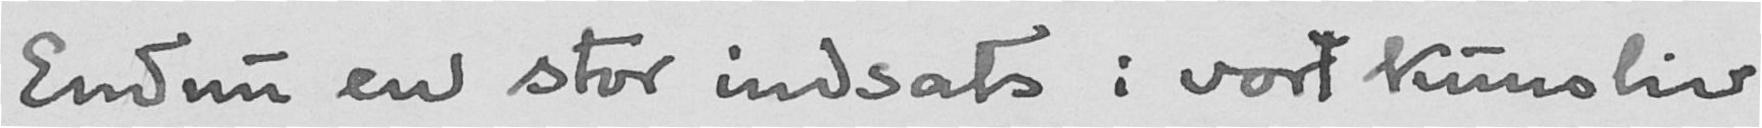Endnu en stor indsats i vort kunstliv
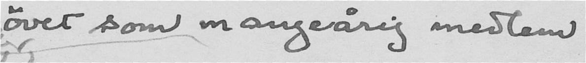øvet som mangeårig medlem
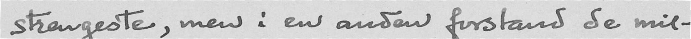stengeste, men i en anden forstand de mil-
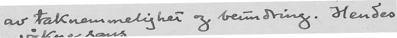av taknemmelighet og beundring. Hendes
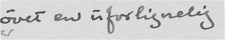øvet en uforlignelig
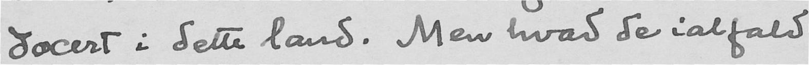docert i dette land. Men hvad de ialfald
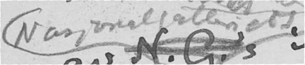Nasjonalgalleriets
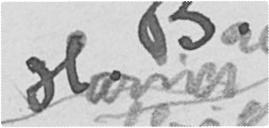Harriet
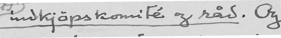indkjøpskomité og råd. Og
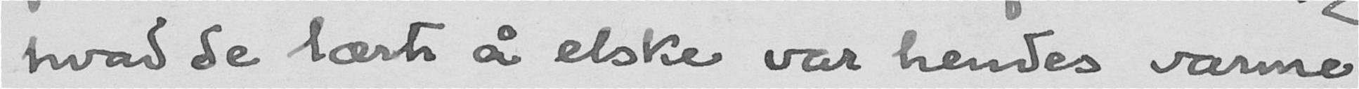hvad de lærte å elske var hendes varme
